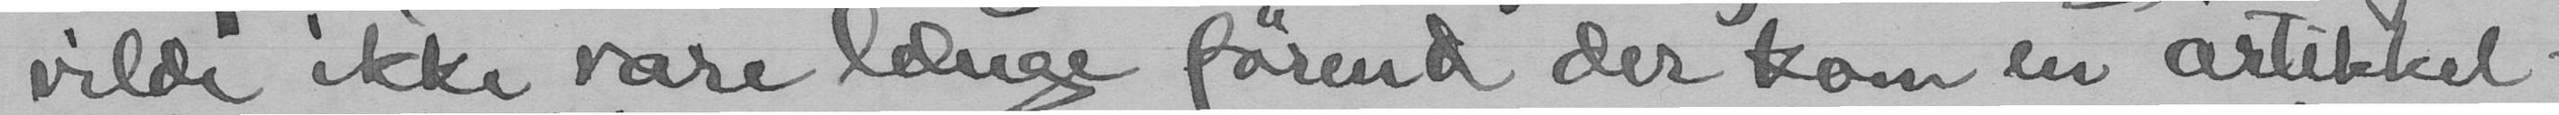vilde ikke være længe førend der kom en artikkel-
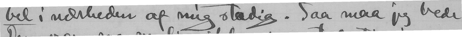bel i nærheden af mig stadig. Saa maa jeg bede
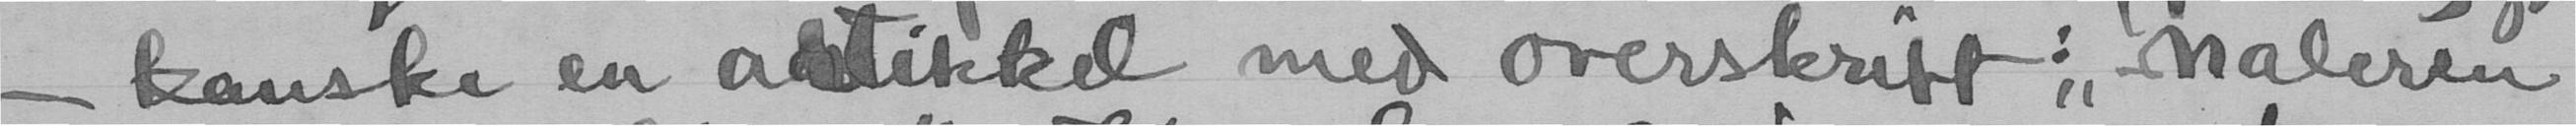- kanske en artikkel med overskrift: "Maleren
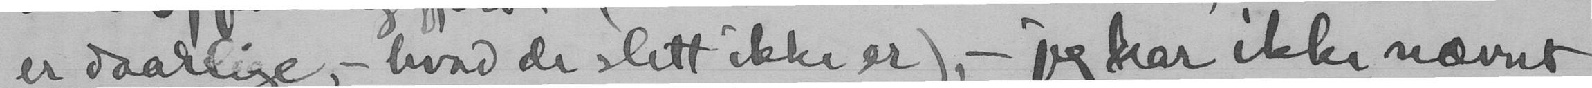er daarlige, - hvad de slett ikke er), - jeg har ikke nævnt
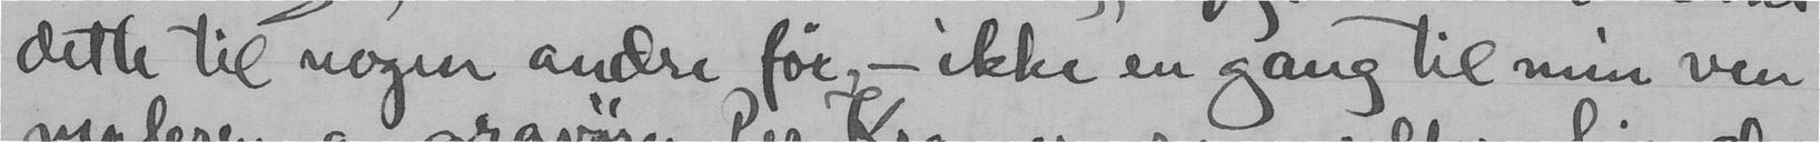dette til nogen andre før, - ikke en gang til min ven
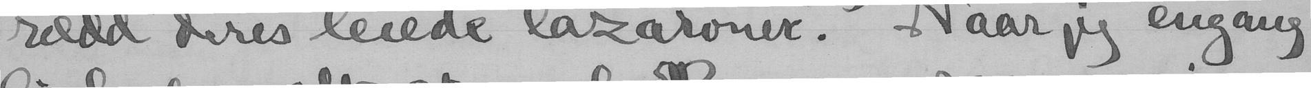rædd deres leiede lazaroner. Naar jeg engang
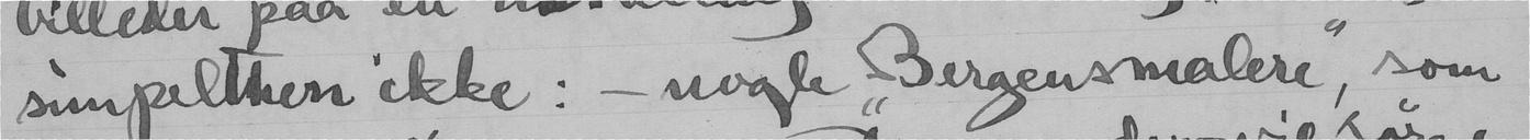simpelthen ikke: - nogle "Bergensmalere", som
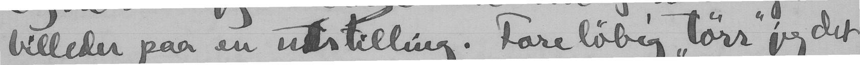billeder paa en udstilling. Foreløbig "tørr" jeg det
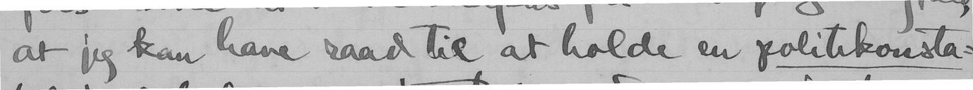at jeg kan have raad til at holde en politekonsta-
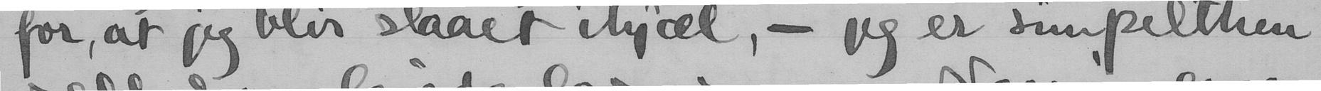for, at jeg blir slaaet ihjæl, - jeg er simpelthen
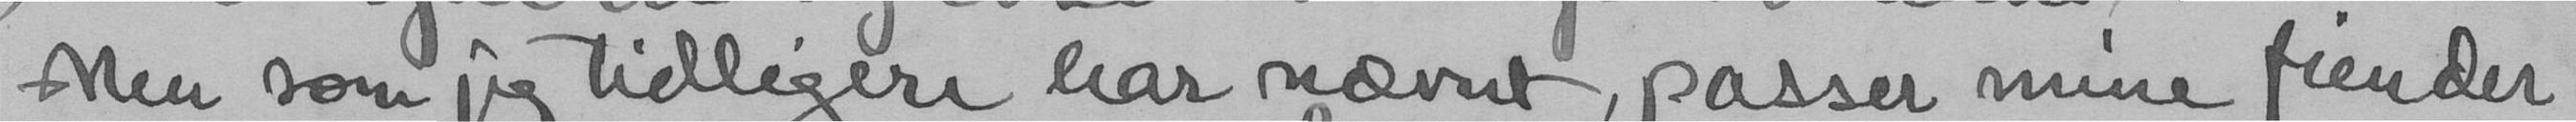Men som jeg tidligere har nævnt, passer mine fiender
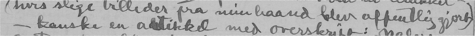(hvis slige billeder fra min haand blev offentlig gjort)
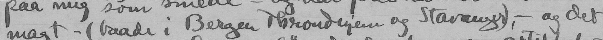magt - (baade i Bergen Throndhjem og Stavanger), - og det
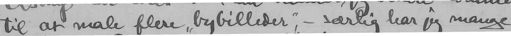til at male flere "bybilleder" - særlig har jeg mange
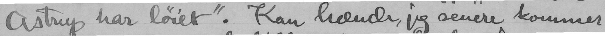Astrup har løiet". Kan hænde, jeg senere kommer
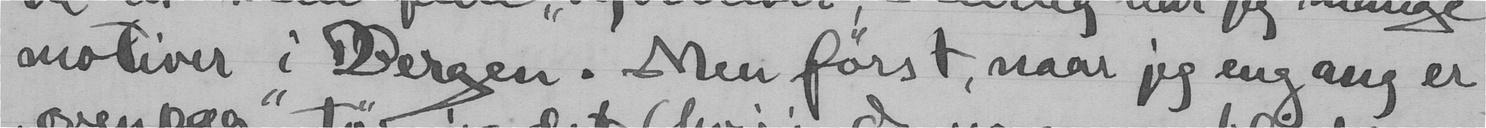motiver i Bergen. Men først, naar jeg engang er
